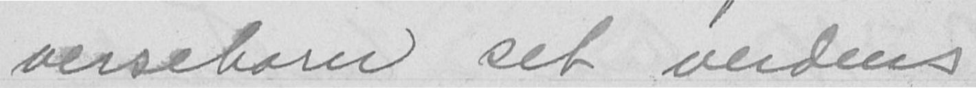versebarn set verdens
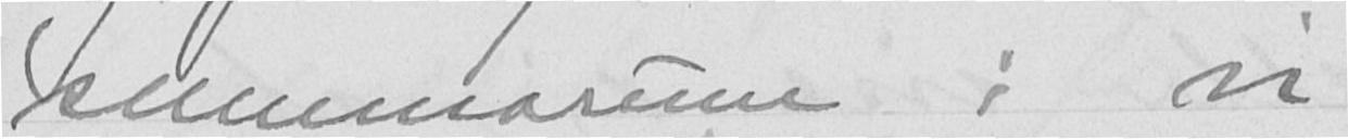summarum: vi
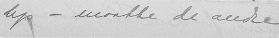lys - maatte de andre
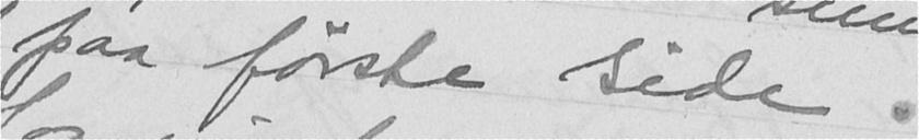paa første side.
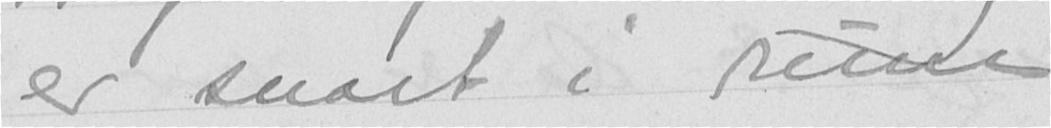er snart i rune
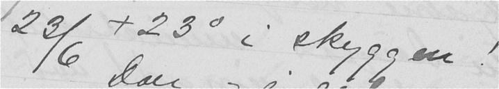23/6 + 23° i skyggen!
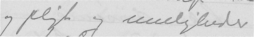og pligt og muligheder
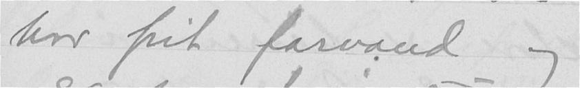har frit farvand og
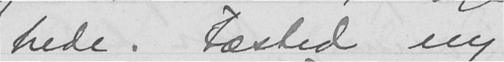hede. Tæsted ny
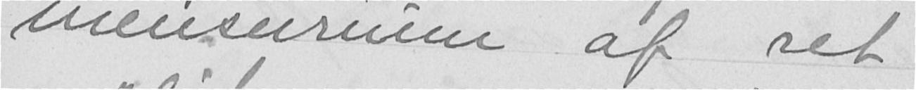mensurium af ret
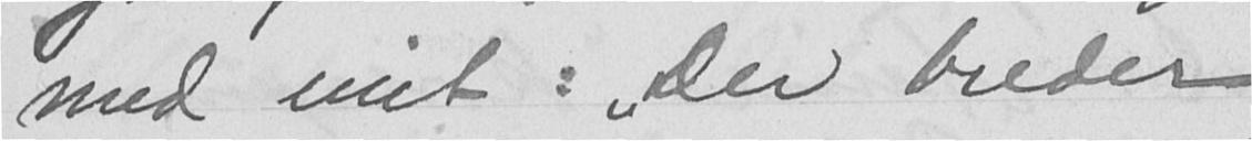med mit: "Der breder
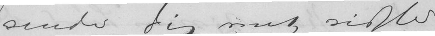sende Dig mit sidste
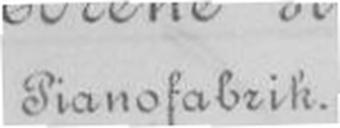Pianobabrik.
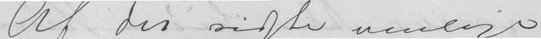Af det sidste venlige
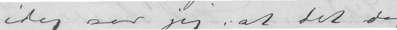idag ser jeg at det dog
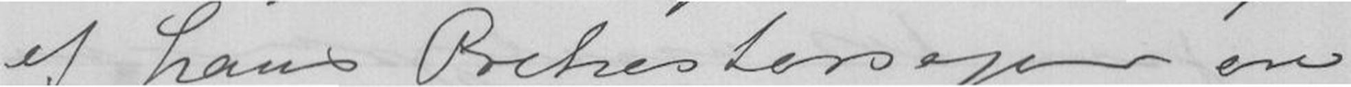af hans Orchestersagen ere
